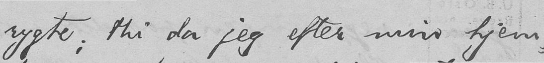rygte; thi da jeg efter min hjem-
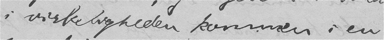i virkeligheden kommen i en
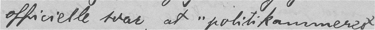officielle svar, at "politikammeret
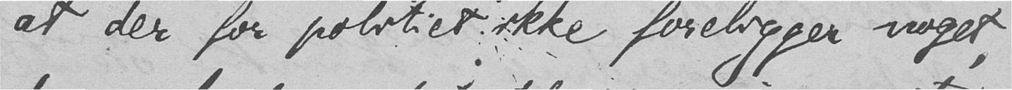at der for politiet ikke foreligger noget,
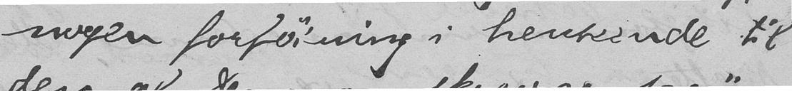nogen forføining i henseende til
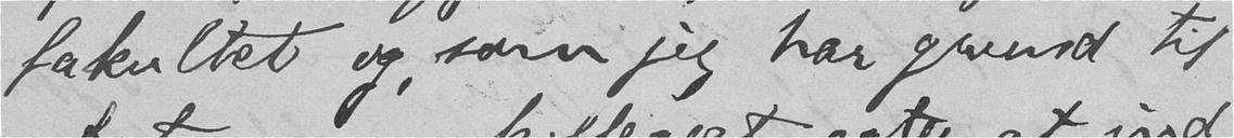fakultet og, som jeg har grund til
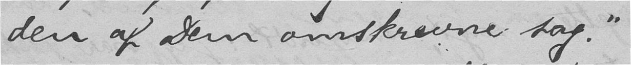den af Dem omskrevne sag."
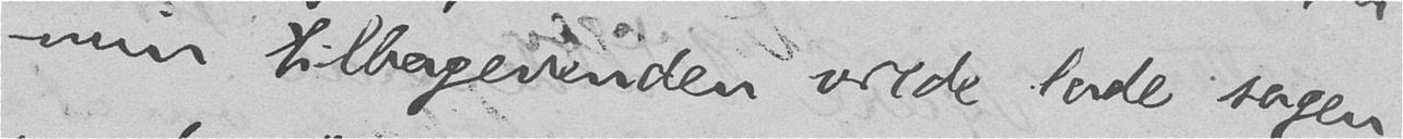min tilbagevenden vilde lade sagen
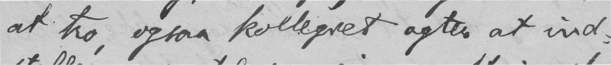at tro, ogsaa kollegiet agter at ind-
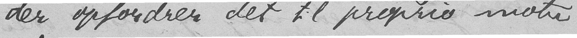der opfordrer det til proprio motu
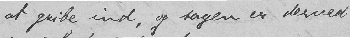at gribe ind, og sagen er derved
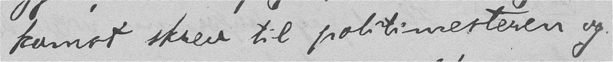komst skrev til politimesteren og
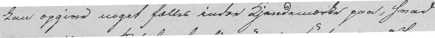kan opgive noget fælles indre Kjendemærke paa, hvad
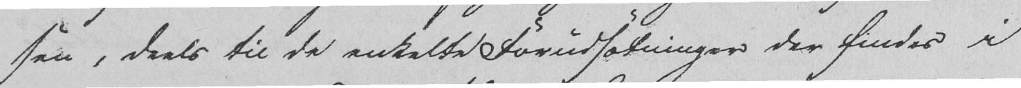sen, deels til de enkelte Forudsætninger der findes i
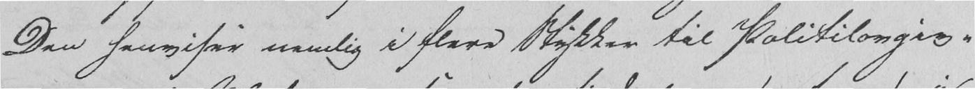Den henviser nemlig i flere Stykker til Politilovgiv-
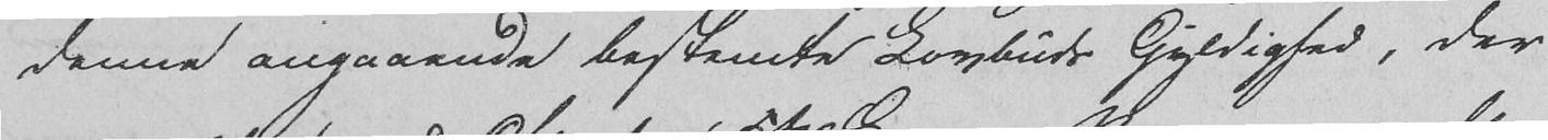denne angaaende bestemte Lovbuds Gyldighed, der
